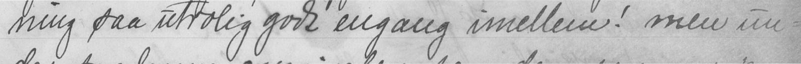ning saa utrolig godt engang imellem! men un-
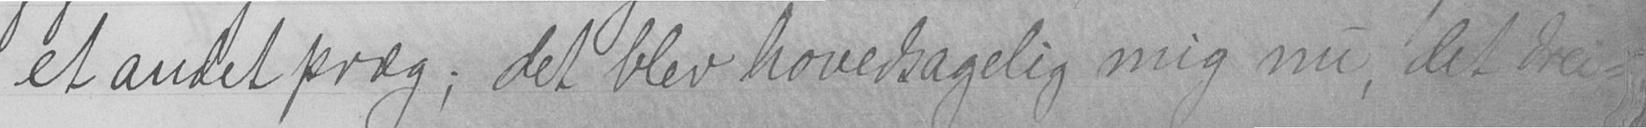et andet præg; det blev hovedsagelig mig nu, det drei-
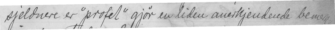sjeldnere er "profet" gjør en liden anerkjendende bemerk-
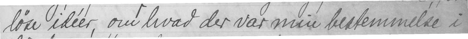løse idéer, om hvad der var min bestemmelse i
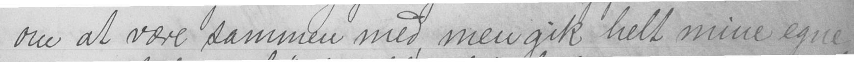om at være sammen med, men gik helt mine egne
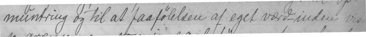muntring og til at faa følelsen af eget værd - inden vis-
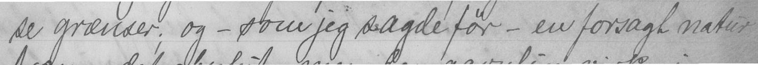se grænser; og - som jeg sagde før - en forsagt natur
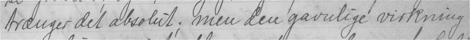trænger det absolut; men den gavnlige virkning
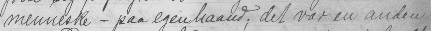menneske - paa egen haand; det var en anden
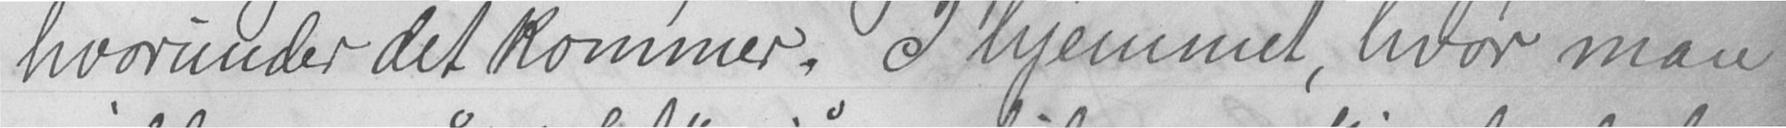hvorunder det kommer. I hjemmet, hvor man
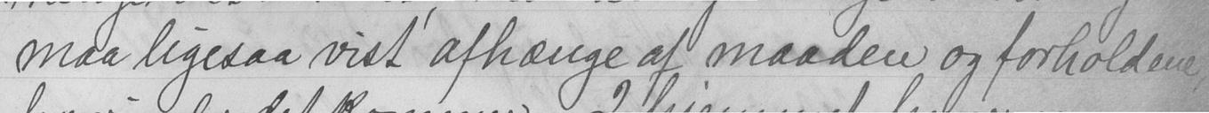maa ligesaa vist afhænge af maaden og forholdene
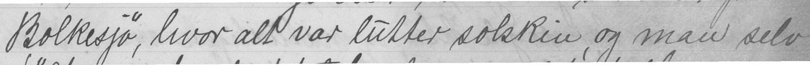Bolkesjø, hvor alt var lutter solskin, og man selv
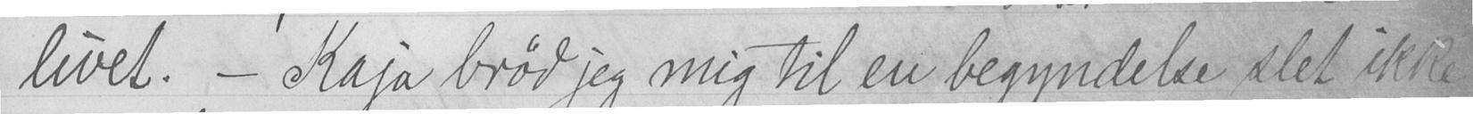livet. - Kaja brød jeg mig til en begyndelse slet ikke
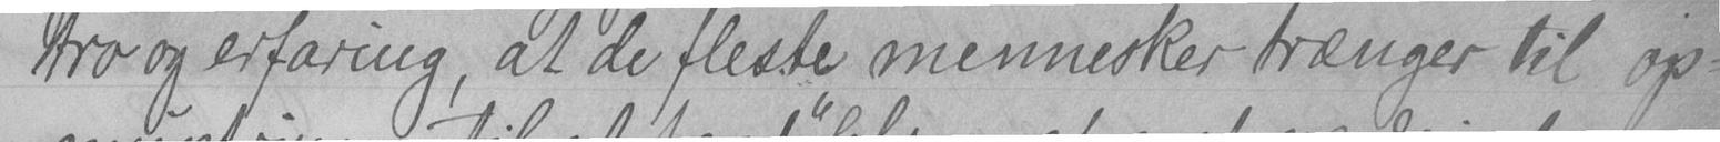tro og erfaring, at de fleste mennesker trænger til op-
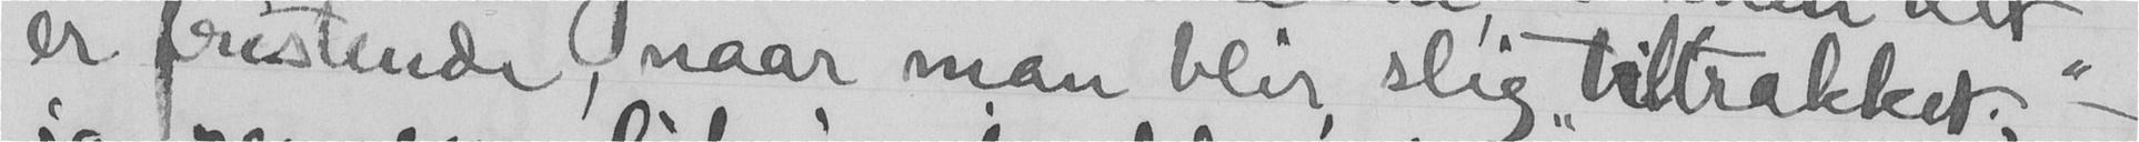er fristende, naar man blir slig "tiltrækket," -
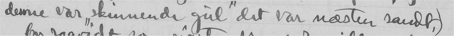denne var "skinnende gul" det var næsten sandt,)
